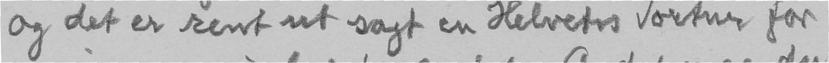og det er rent ut sagt en Helvetes Tortur for
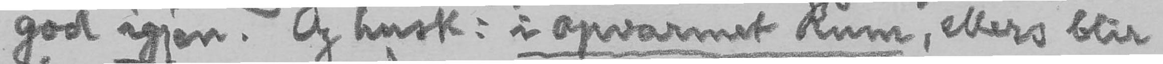god igjen. Og husk: i opvarmet Rum, ellers blir
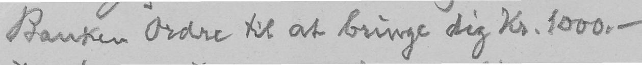Banken Ordre til at bringe dig Kr. 1000. -
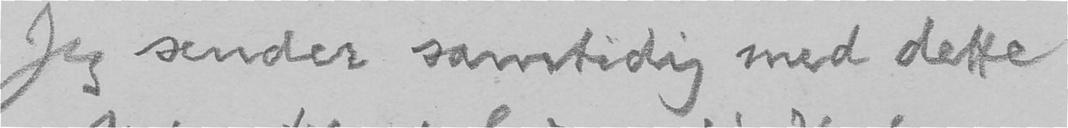Jeg sender samtidig med dette
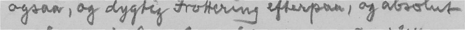ogsaa, og dygtig Frottering efterpaa, og absolut
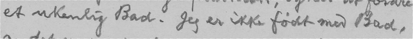et ukenlig Bad. Jeg er ikke født med Bad,
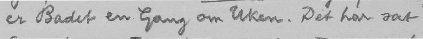er Badet en Gang om Uken. Det har sat
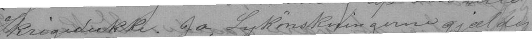Skrigedukke. Ja, Lykønskningerne gjælder
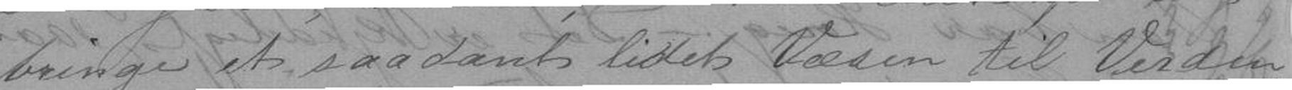bringe et saadant lidet Væsen til Verden,
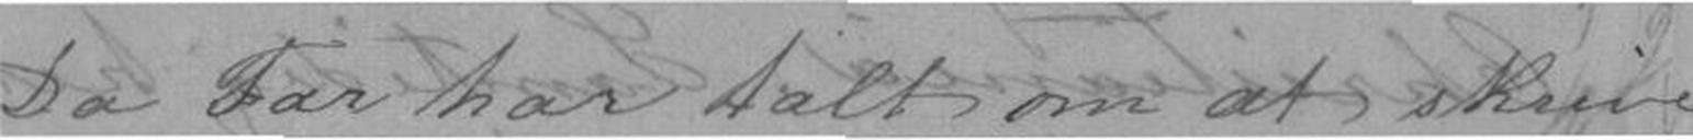Da Far har talt om at skrive
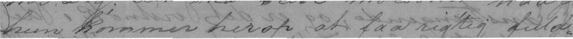hun kommer herop, at faa rigtig fuld-
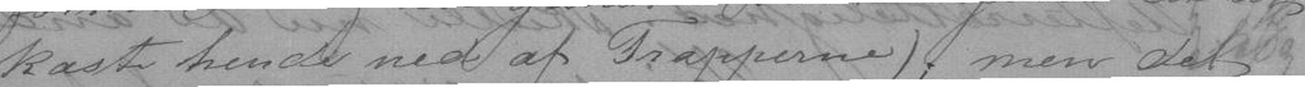kaste hende ned af Trapperne), men det
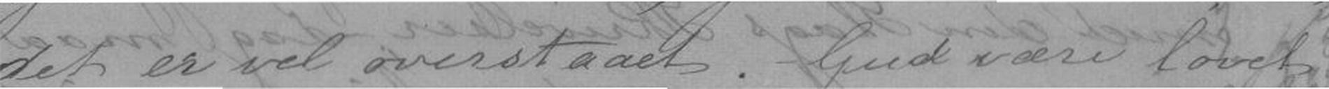det er vel overstaaet. Gud være lovet
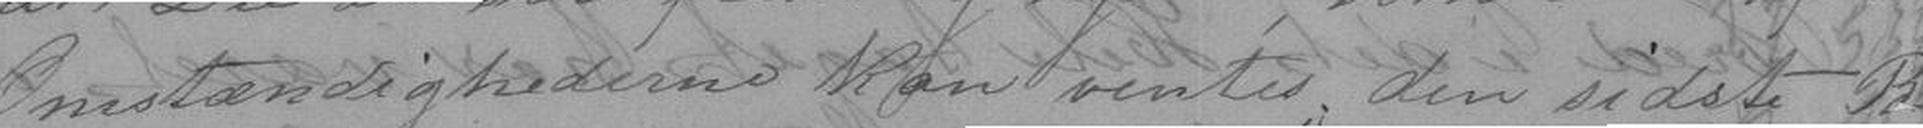Omstændighederne kan ventes, den sidste Be-
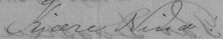Kjære Nina!
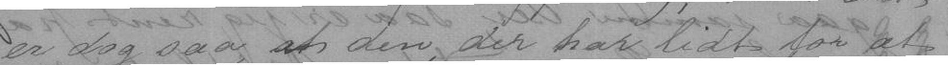er dog saa, at den, der har lidt for at
